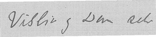Vislie og Dem selv
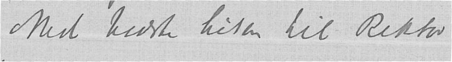Med bedste hilsen til Rektor
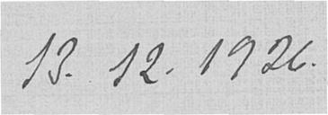13.12.1926.
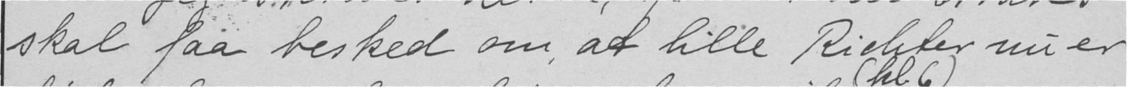skal faa besked om at lille Richter nu er
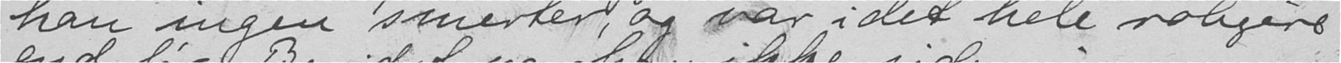han ingen smerter, og var i det hele roligere
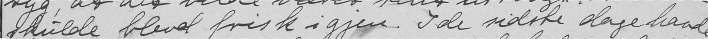skulde blevet frisk igjen. I de sidste dage havde
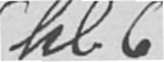(kl 6)
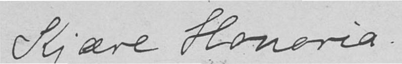Kjære Honoria.
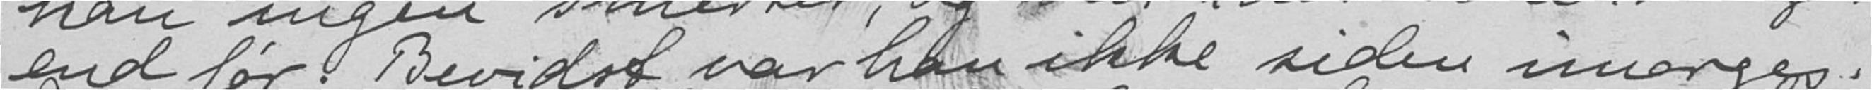end før. Bevidst var han ikke siden imorges.
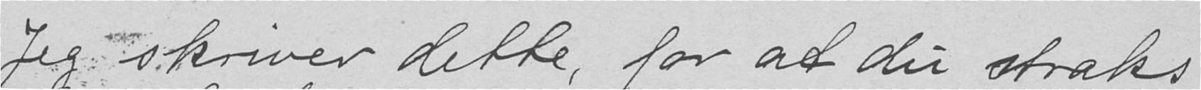Jeg skriver dette, for at du straks
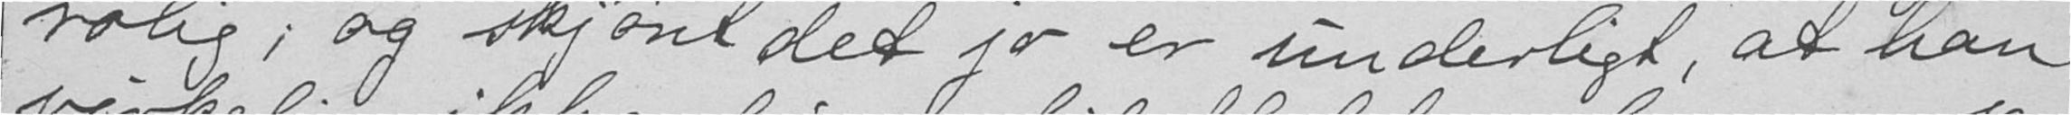rolig; og skjønt det jo er underligt, at han
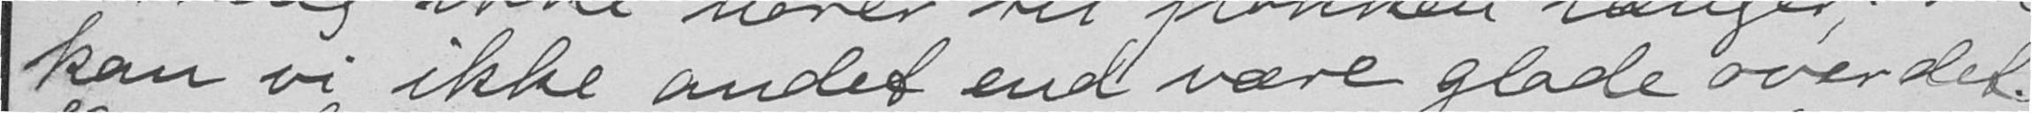kan vi ikke andet end være glade over det.
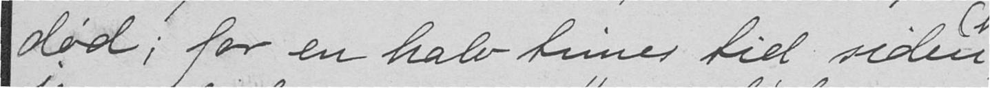død; for en halv times tid siden,
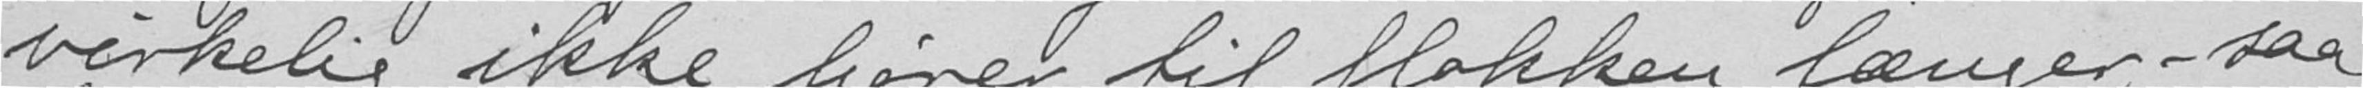virkelig ikke hører til flokken længer - saa
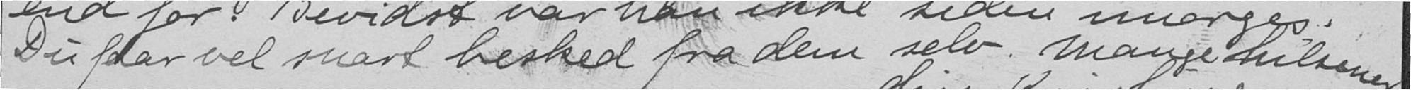Du faar vel snart besked fra dem selv. Mange hilsener
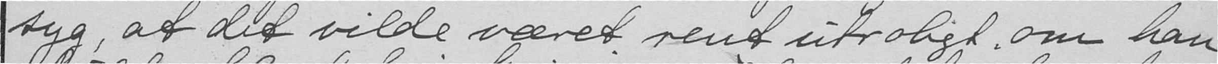syg, at det vilde været rent utroligt, om han
